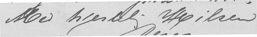Med hjertelig Hilsen
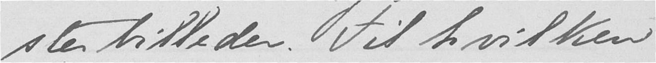sterbilleder. Til hvilken
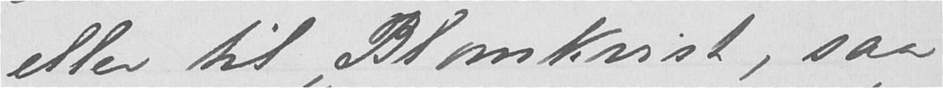eller til Blomkvist, saa
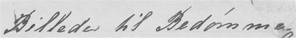Billeder til Bedømme.
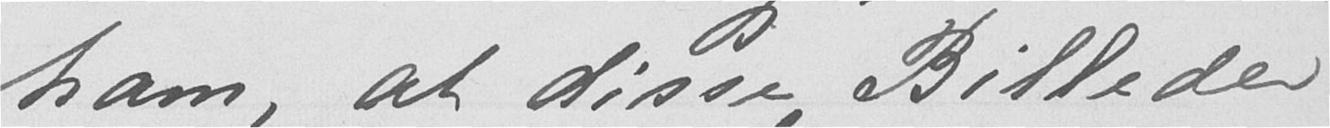ham, at disse Billeder
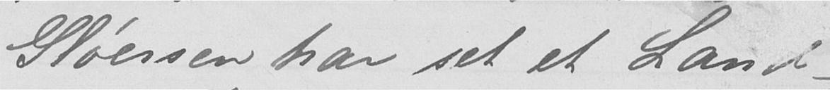Gløersen har set et Land-
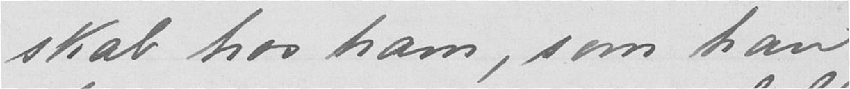skab hos ham, som han
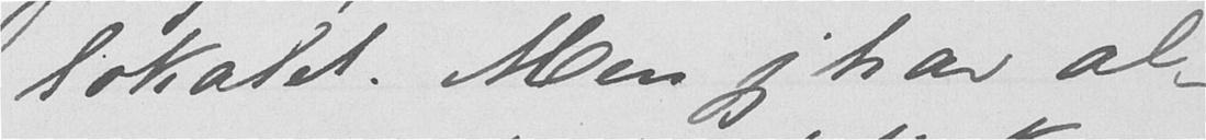lokalet. Men jeg har al-
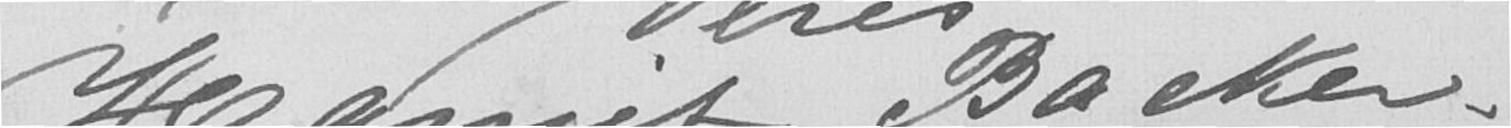Harriet Backer.
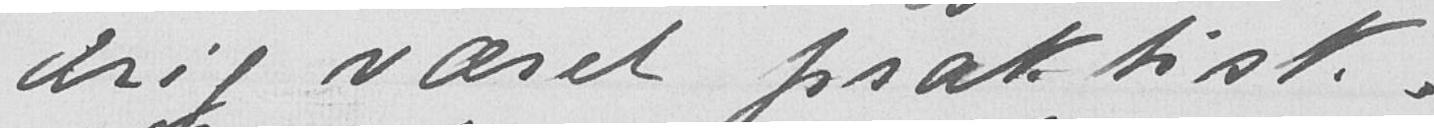drig været praktisk.
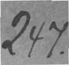247.
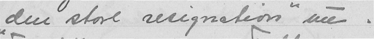"den store resignation" nu.
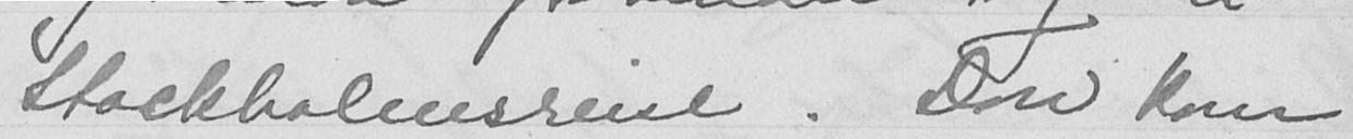Stockholmsreise. Don kom
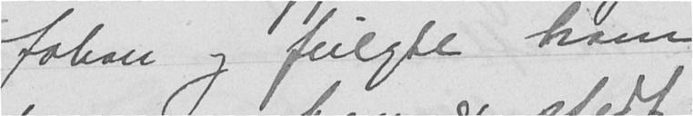Johan og fulgte ham
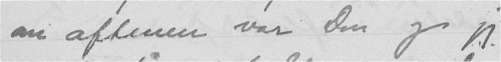om aftenen var Don og jeg
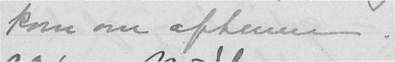kom om aftenen.
